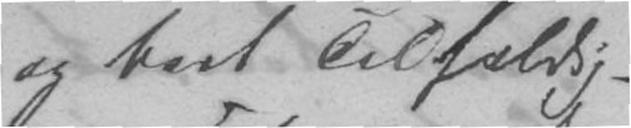og bart Tilfældig-
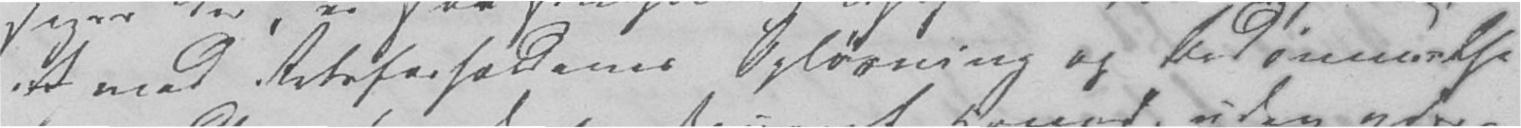med Retsforholdenes Opløsning og Bedømmelse
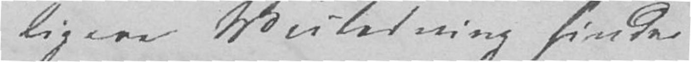ligere Veiledning finder
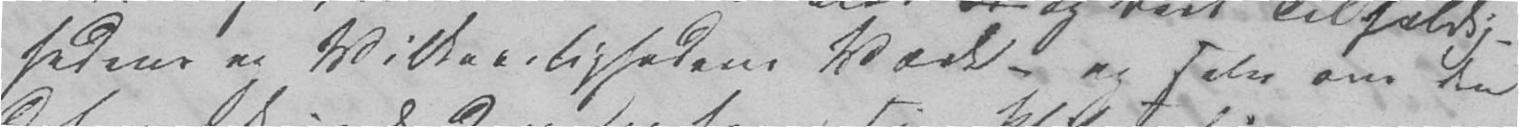hedene og Vilkaarlighedens Værk – og selv om den
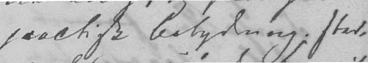practisk Betydning sted-
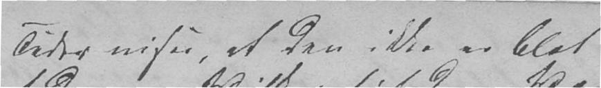Tider viser, at den ikke er blot
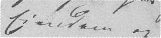Eiendom og
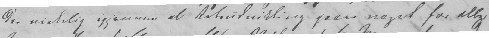der virkelig igjennem al Retsudvikling gaaer noget for alle
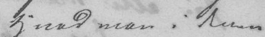Hvad man i denne
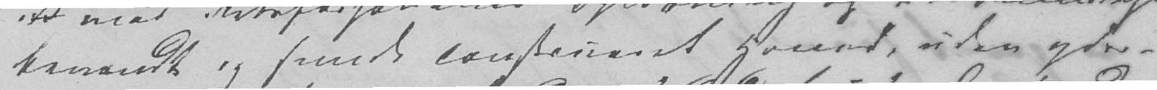bevandt og sundt construeret Hoved, uden yder-
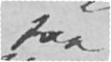faa
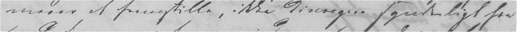maaer at fremstille, ikke divergere syndeligt fra
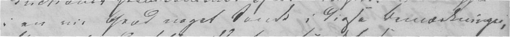i en vis Grad noget Sandt i disse Bemærkninger,
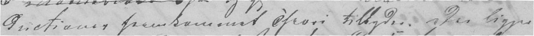ductioner fremkommet Theori tilbyder. Der ligger
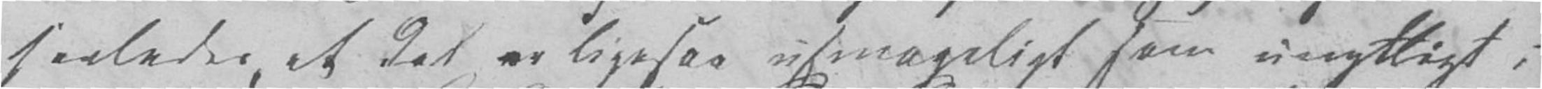saaledes, at det er ligesaa usmageligt som unyttigt i
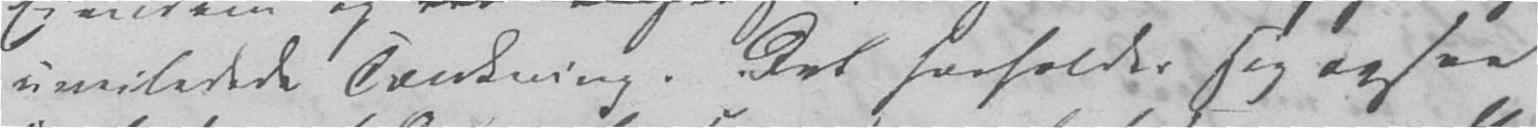uveiledede Tænkning. Det forholder sig ogsaa
